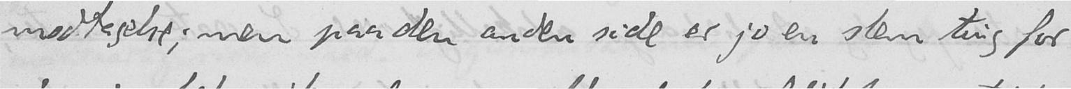modtagelse; men paa den anden side er jo en slem ting for
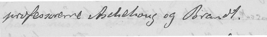professorerne Aschehoug og Brandt.
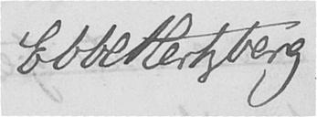Ebbe Hertzberg.
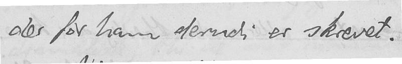der før ham dermedi er skrevet.
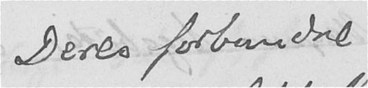Deres forbundne
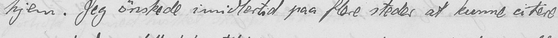hjem. Jeg ønskede imidlertid paa flere steder at kunne citere
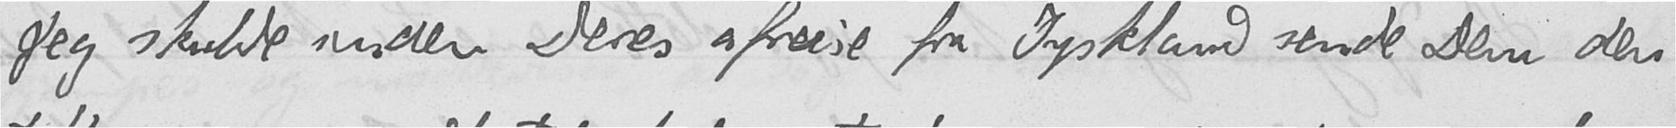Jeg skulde inden Deres afreise fra Tyskland sende Dem den
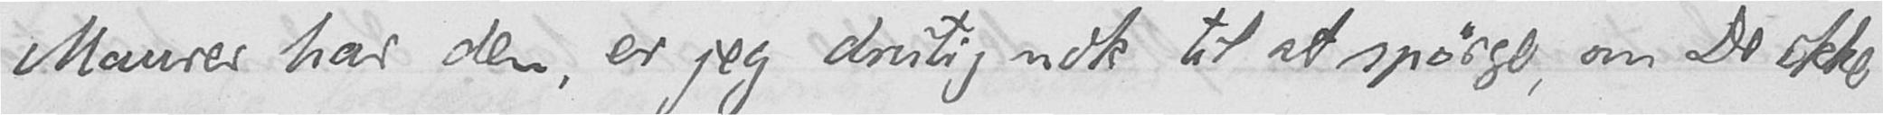Maurer har den, er jeg dristig nok til at spørge, om De ikke
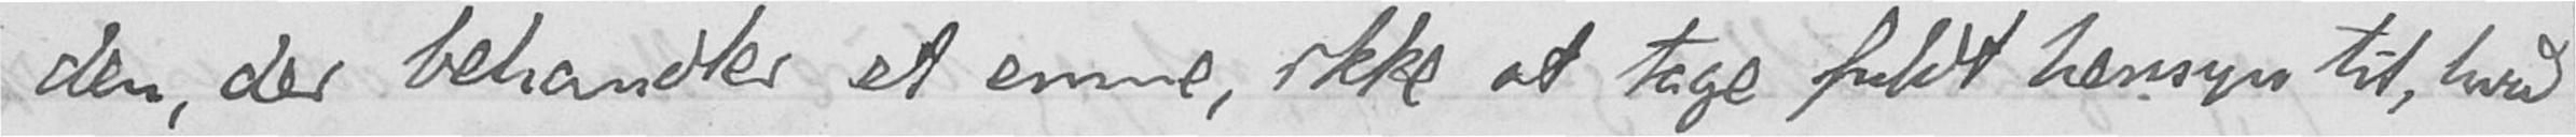den, der behandler et emne, ikke at tage fuldt hensyn til, hvad
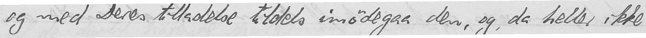og med Deres tilladelse tildels imødegaa den, og, da heller ikke
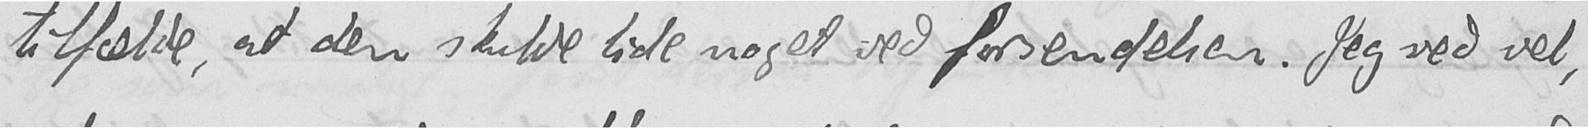tilfælde, at den skulde lide noget ved forsendelsen. Jeg ved vel,
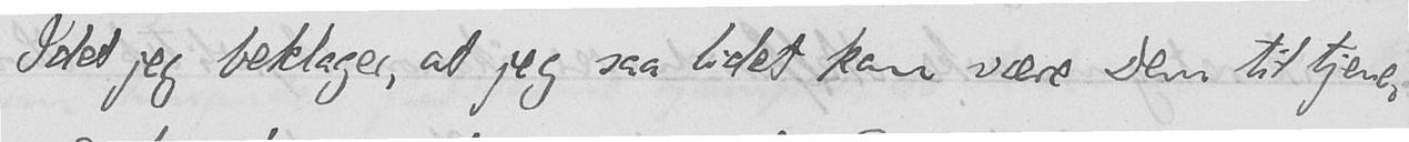Idet jeg beklager, at jeg saa lidet kan være Dem til tjene-
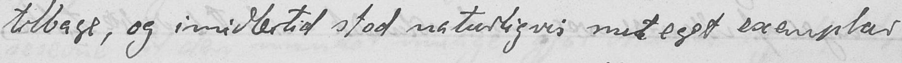tilbage, og imidlertid stod naturligvis mit eget exemplar
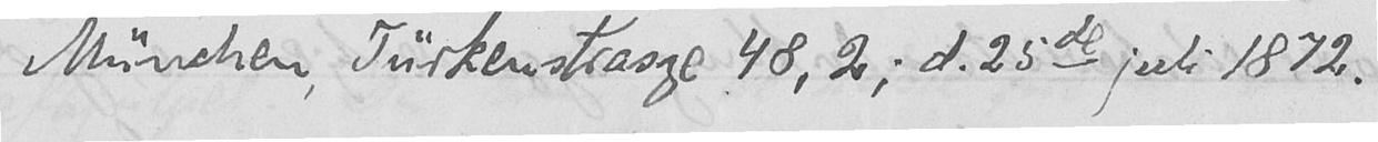München, Türkenstrasse 48, 2; d. 25de juli 1872.
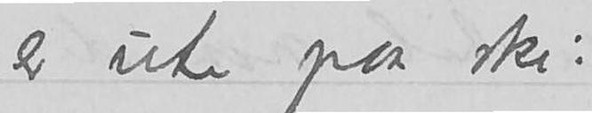er ute på ski.
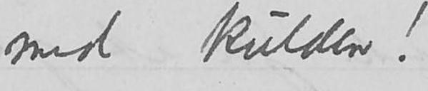med kulden!
